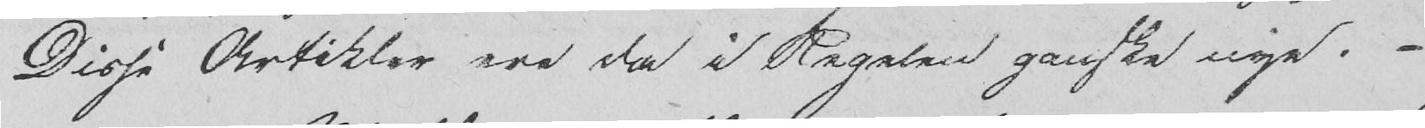Disse Artikler ere da i Regelen ganske nye. -
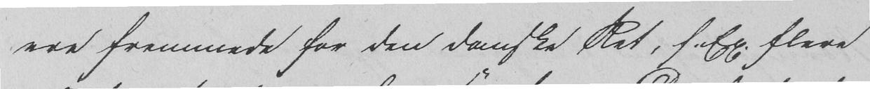ere fremmede for den danske Ret, f. Ex. flere
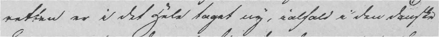retten er i det Hele taget ny, ialfald i den danske
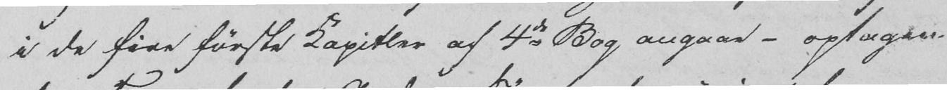i de fire første Kapitler af 4de Bog angaar - optagen
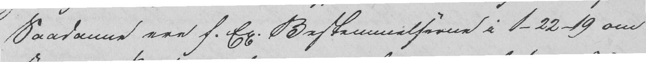Saadanne ere f. Ex. Bestemmelserne i 1-22-19 om
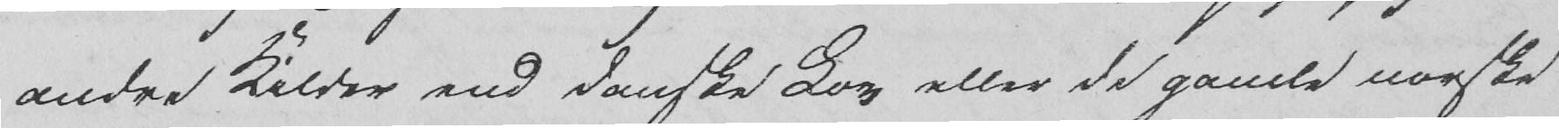andre Kilder end danske Lov eller de gamle norske
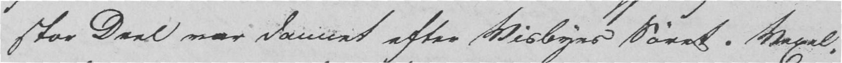stor Deel var dannet efter Visbyes Søret. Vexel-
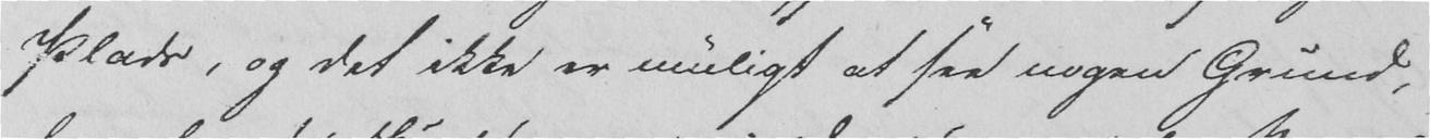Plads, og det ikke er muligt at see nogen Grund,
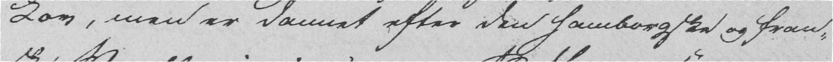Lov, men er dannet efter den hamborgske og fran-
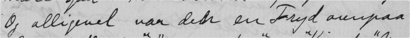Og alligevel var den en Fryd ovnpaa
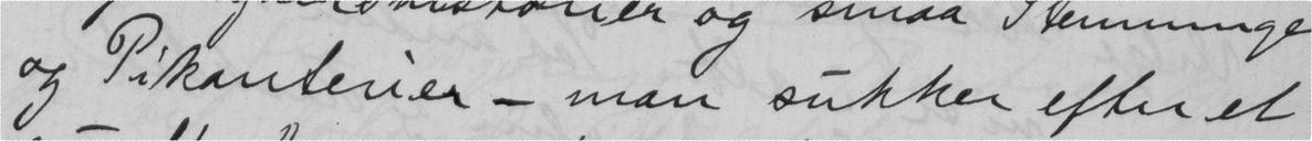og Pikanterier - man sukker efter et
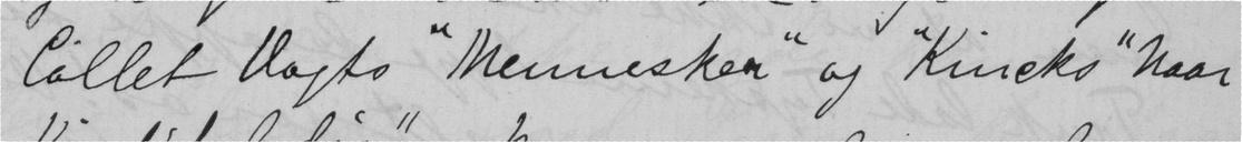Collet Vogts "Mennesker" og Kincks "Naar
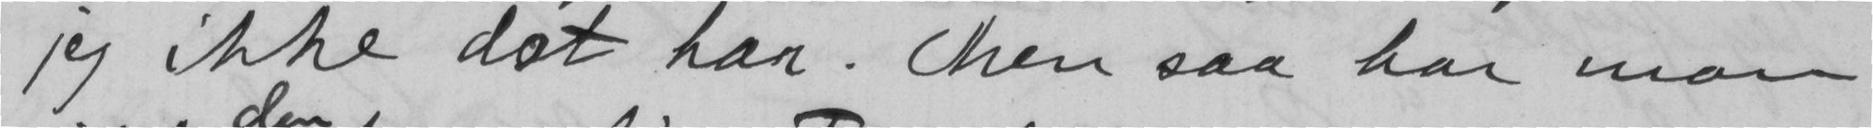jeg ikke det har. Men saa har mon
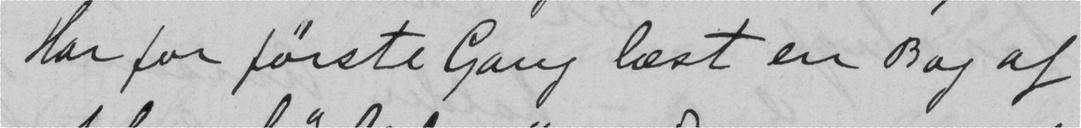Har for første Gang læst en Bog af
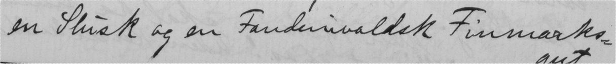en Slusk og en Fandenivoldsk Finmarks-
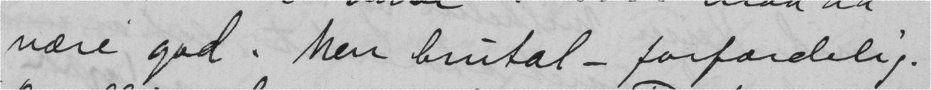være god. Men brutal - forfærdelig.
